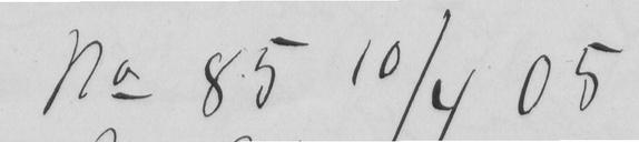No 85 10/4 05
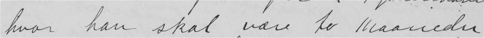hvor han skal være to Maaneder
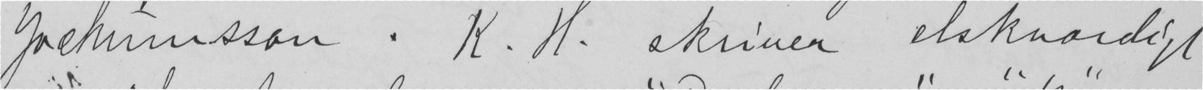Jochumsson. K. H. skriver elskværdigt
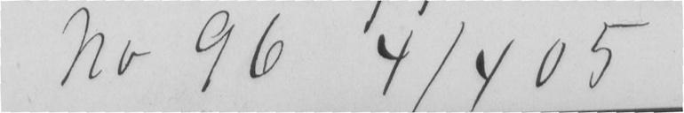N6 96 4/4 05
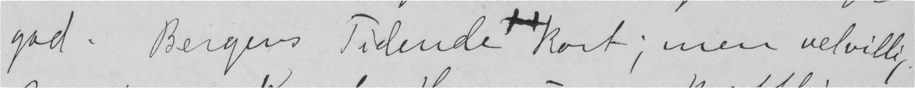god. Bergens Tidende Kort; men velvillig.
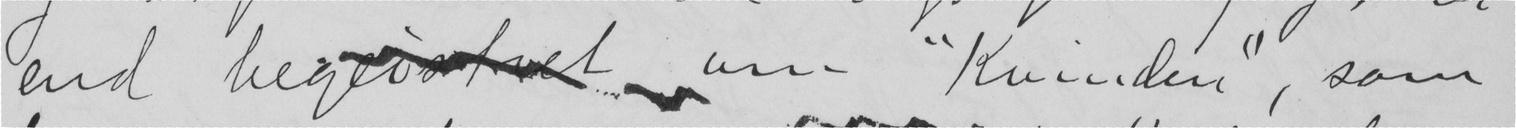end begeistret om "Kvinden", som
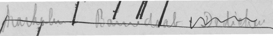Marholm Barnedaab Dedichen
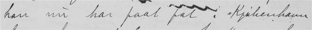han nu har faat fat i Kjøbenhavn
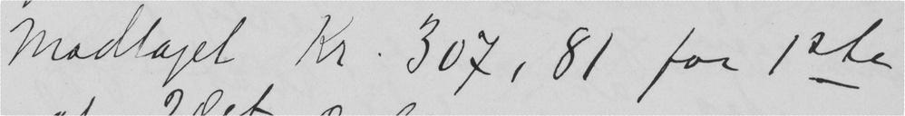Modtaget Kr. 307, 81 for 1ste
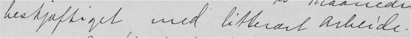bestjæftiget med litterært arbeide.
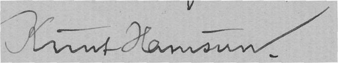Knut Hamsun.
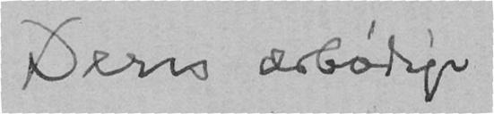Deres ærbødige
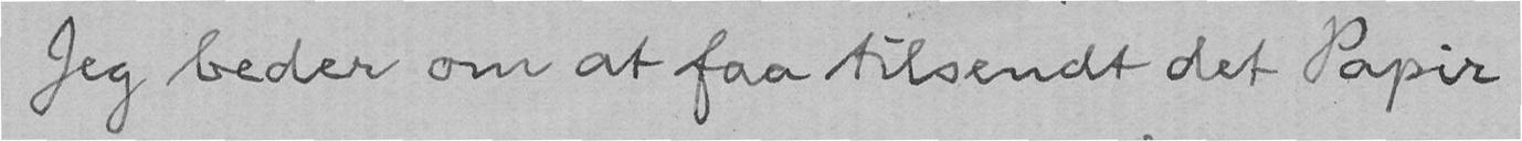Jeg beder om at faa tilsendt det Papir
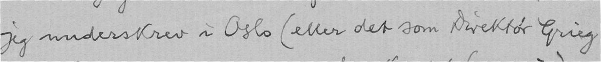jeg underskrev i Oslo (eller det som Direktør Grieg
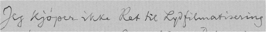Jeg kjøper ikke Ret til Lydfilmatisering
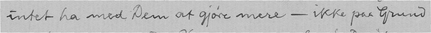intet ha med Dem at gjøre mere - ikke paa Grund
